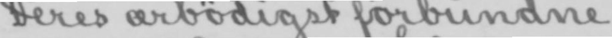Deres ærbødigst forbundne
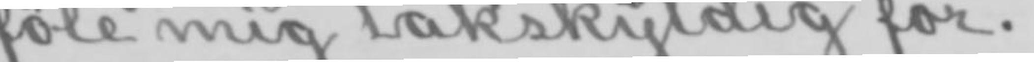føle mig takskyldig for.
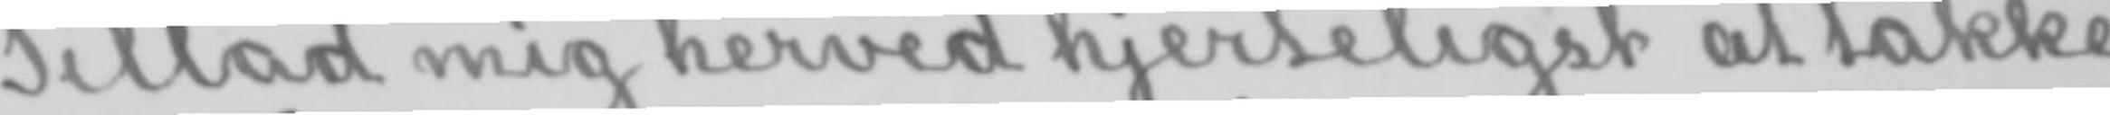Tillad mig herved hjerteligst at takke
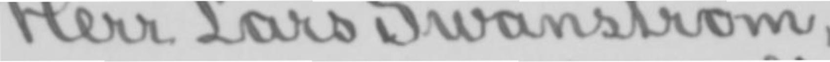Herr Lars Swanstrøm,
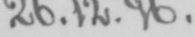26.12.96.
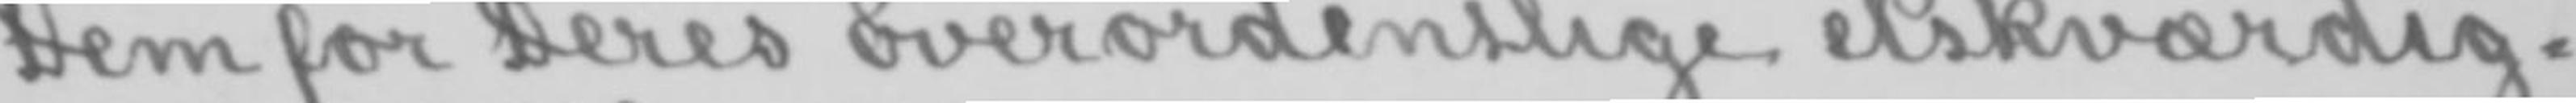Dem for Deres overordentlige elskværdig-
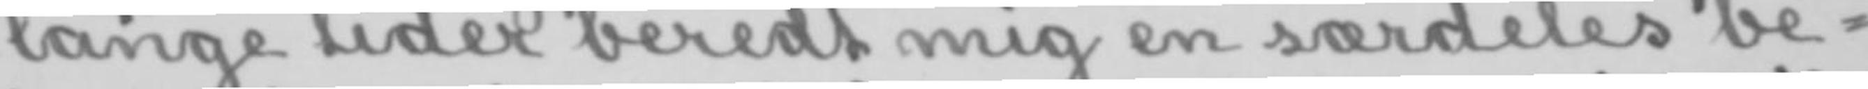lange tider beredt mig en særdeles be-
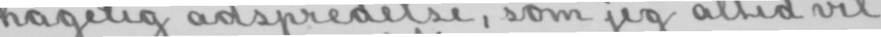hagelig adspredelse, som jeg altid vil
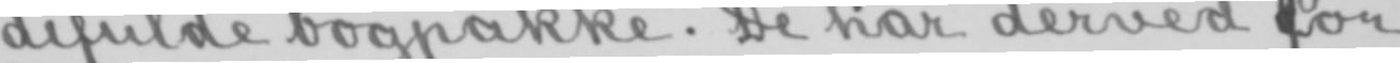difulde bogpakke. De har derved for
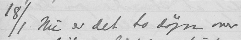18/1 Nu er det to døgn over
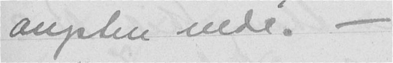angsten inde. -
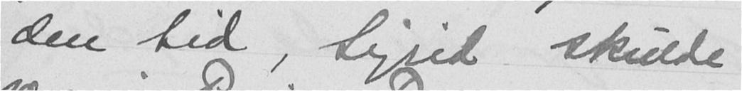den tid, Sigrid skulde
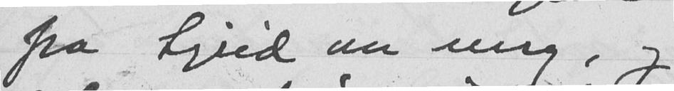fra Sigrid om mrg, og
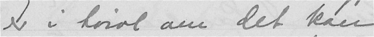er i tvivl om det kan
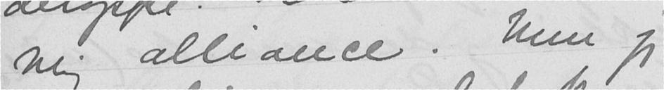mig alliance. Men jeg
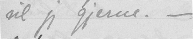vil jeg gjerne. -
